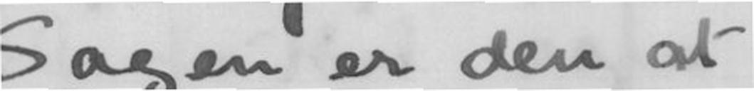Sagen er den at jeg
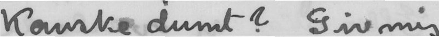kanske dumt? Giv mig
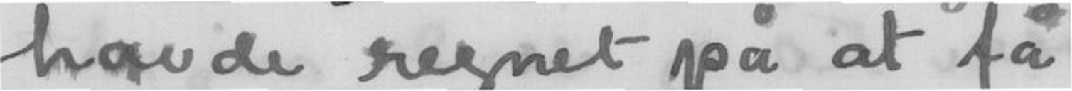havde regnet på at få
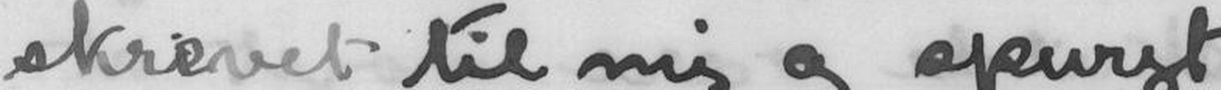skrevet til mig og spurgt
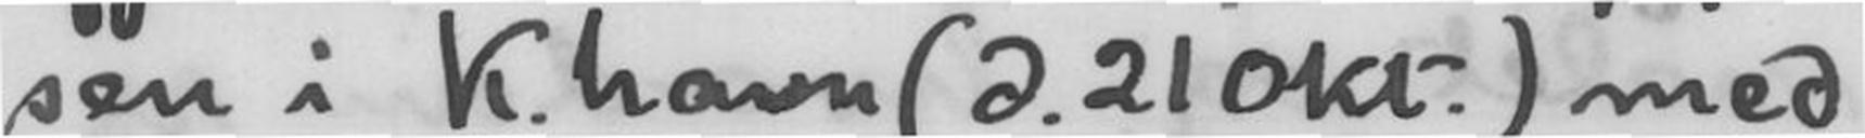sen i K.havn (d.21 okt.) med
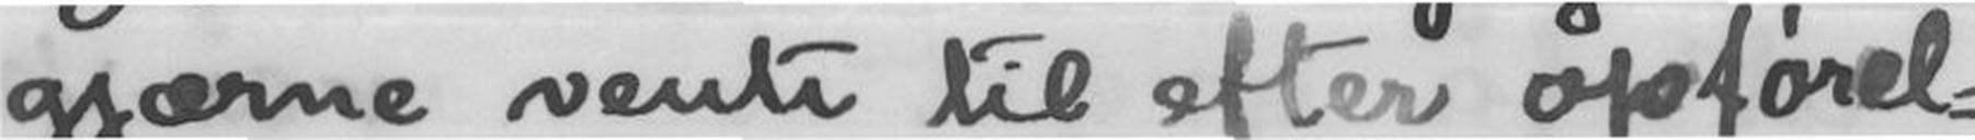gjærne vente til efter opførel-
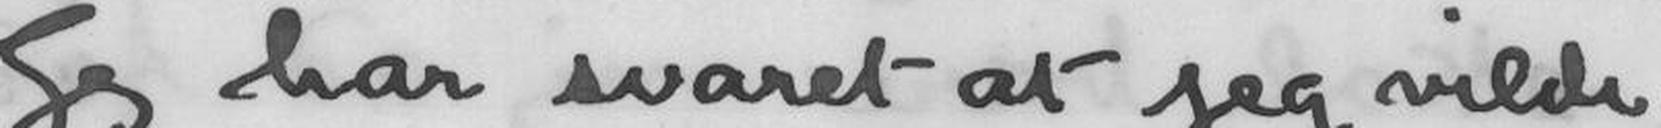Jeg har svaret at jeg vilde
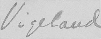Vigeland
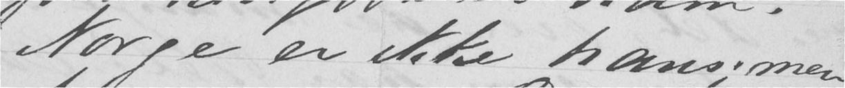Norge er ikke hans; men
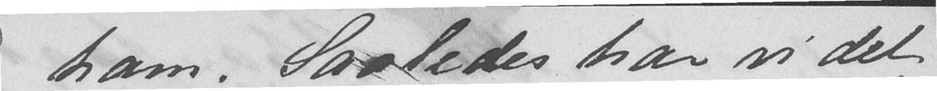ham. Saaledes har vi det
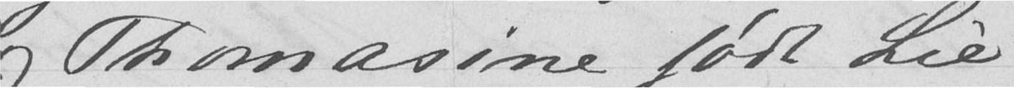og Thomasine født Lie.
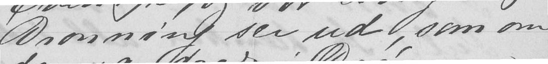Dronning ser ud, som om
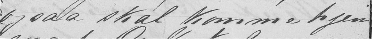ogsaa skal komme hjem
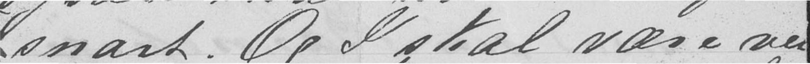snart. Og I skal være vel-
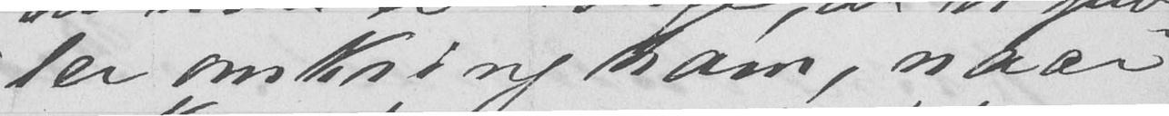ler omkring ham, naar
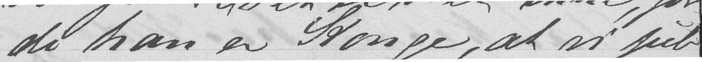di han er Konge, at vi jub-
